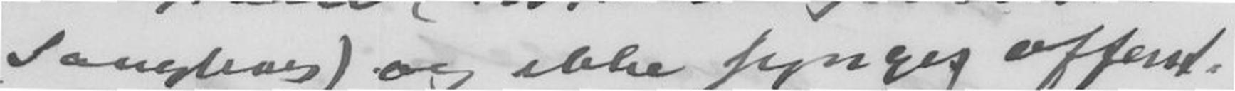Sangbog) og ikke synges offent-
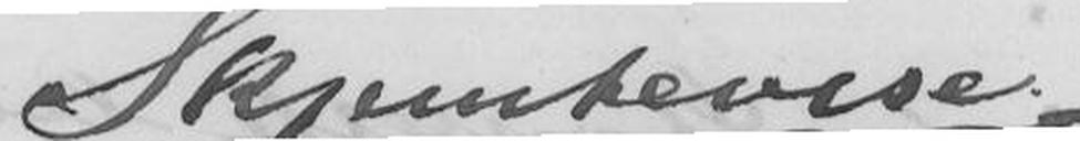Skjemtevise
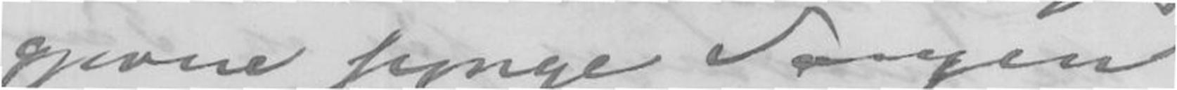gjerne synge Sangen
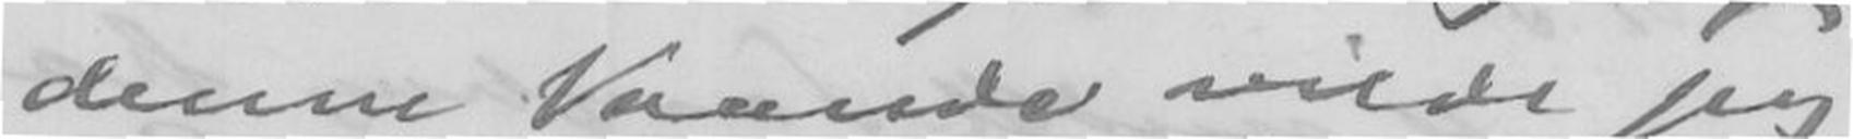denne Vaande vilde jeg
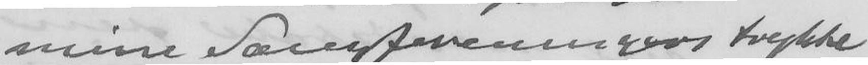mine Sangforeningers trykte
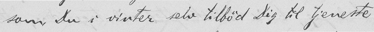som Du i vinter selv tilbød Dig til tjeneste
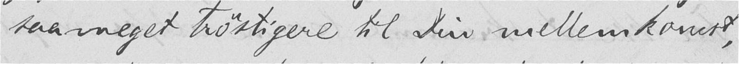saa meget trøstigere til Din mellemkomst,
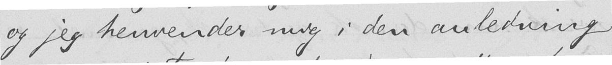og jeg henvender mig i den anledning
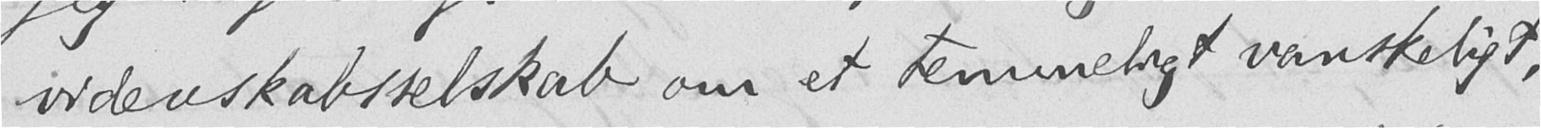videnskabsselskab om et temmeligt vanskeligt,
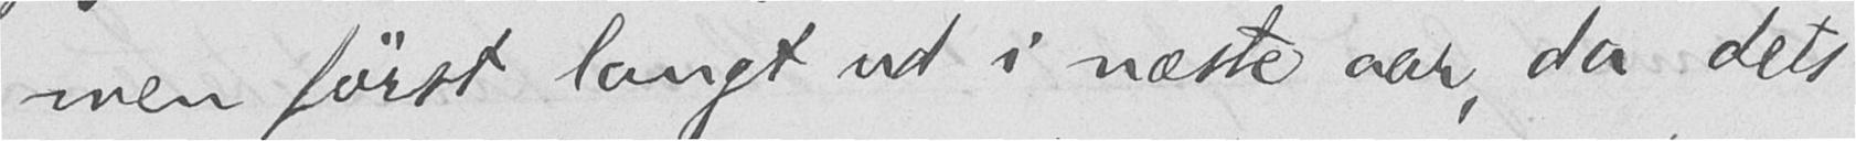men først langt ud i næste aar, da dets
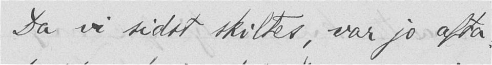Da vi sidst skiltes, var jo afta-
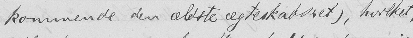kommende den ældste ægteskabsret), hvilket,
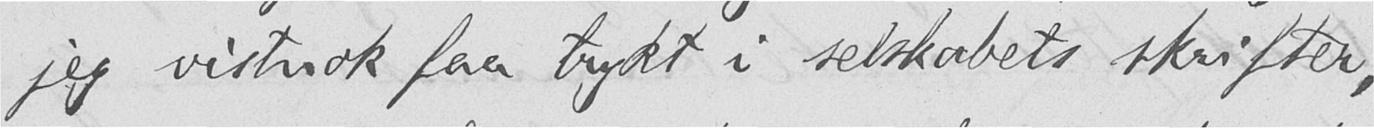jeg vistnok faa trykt i selskabets skrifter,
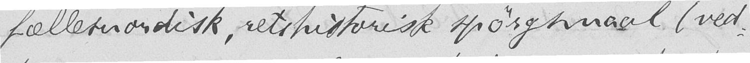fællesnordisk, retshistorisk spørgsmaal (ved-
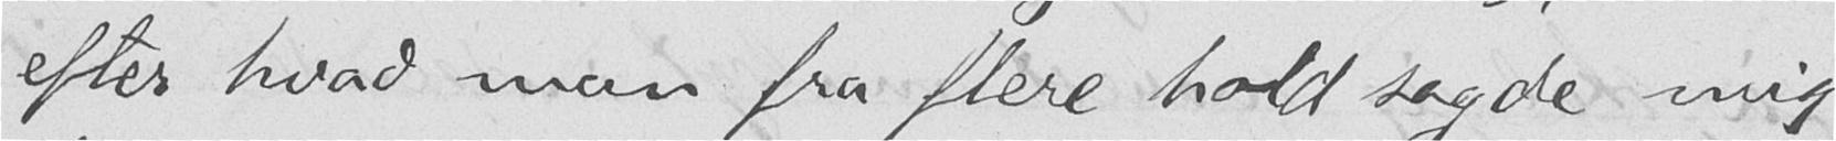efter hvad man fra flere hold sagde mig,
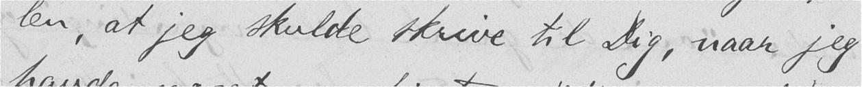len, at jeg skulde skrive til Dig, naar jeg
